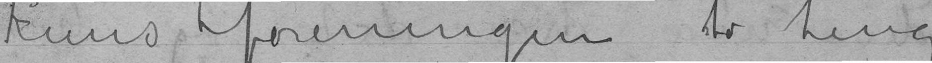Kunstforeningen to ting
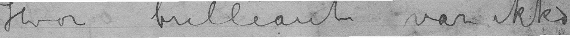Hvor brilliant var ikke
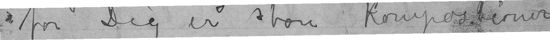for Dig er store Kompositioner
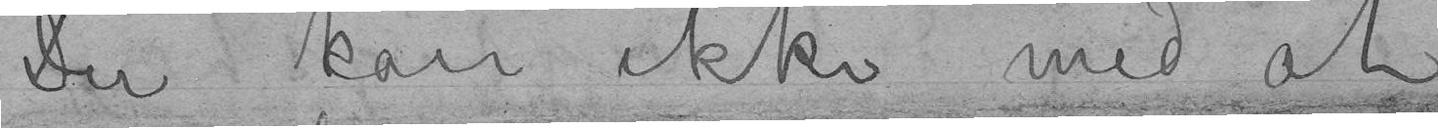Du kan ikke med at
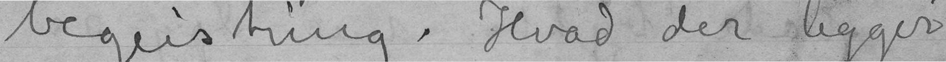begeistring. Hvad der ligger
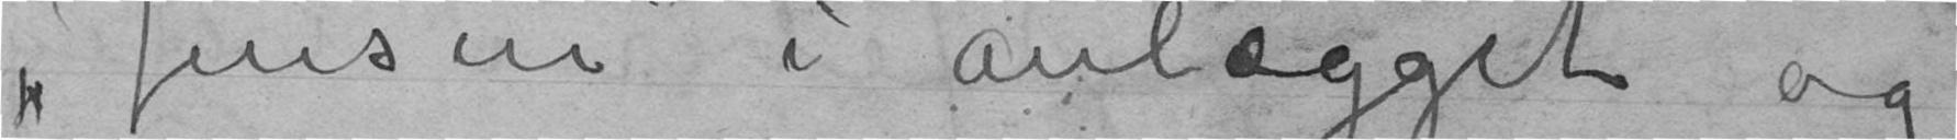«Jensen» i anlægget og
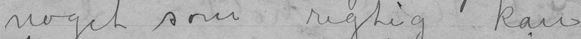noget som rigtig kan
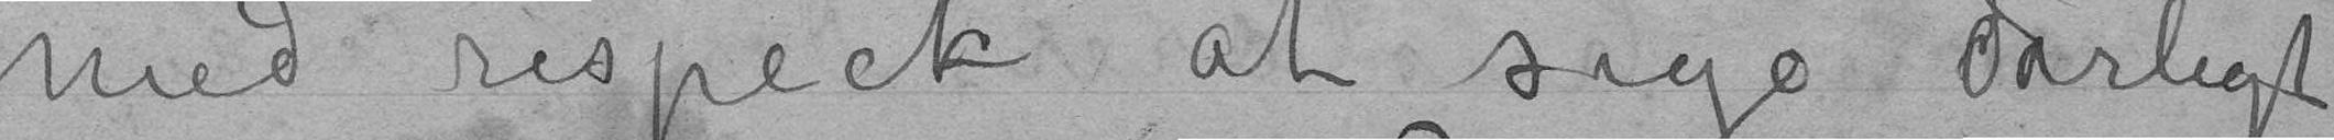med respeckt at sige dårligt
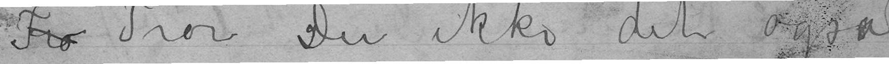Tror Du ikke det også
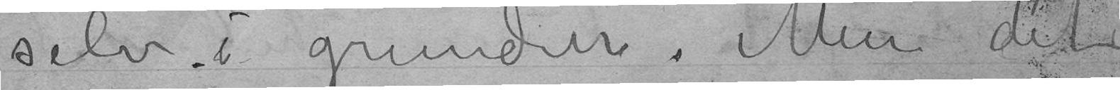selv i grunden. Men det
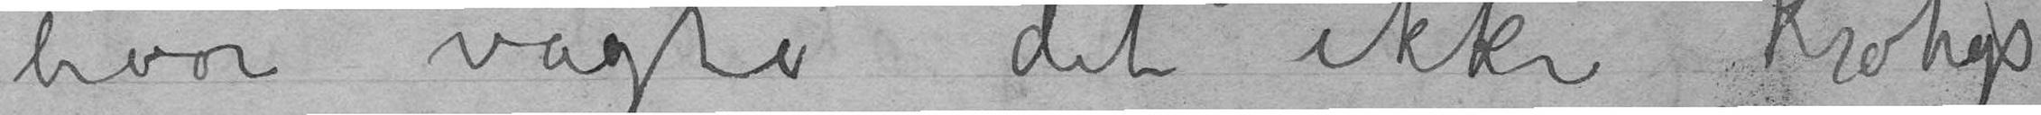hvor vagte det ikke Krohgs
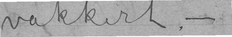vakkert. –
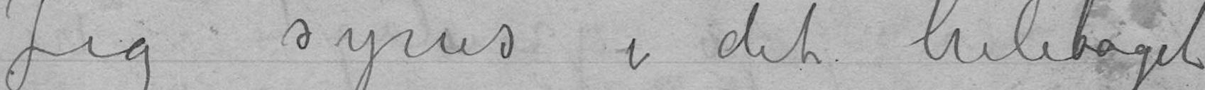Jeg synes i det heletaget
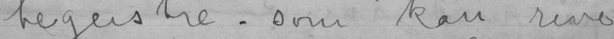begeistre – som kan rive
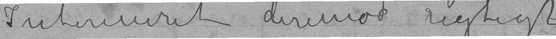Interieuret derimod rigtigt
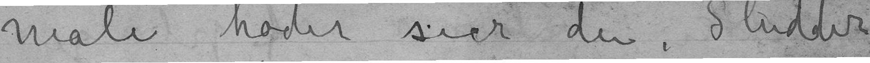male hoder sier du. Sludder
